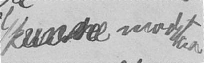kunde modstaa
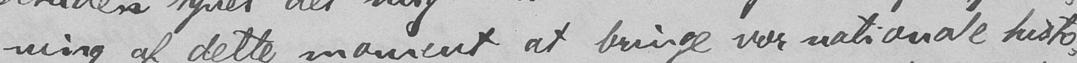ning af dette moment, at bringe vor nationale histo-
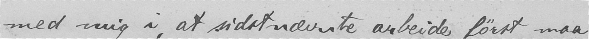med mig i, at sidstnævnte arbeide først maa
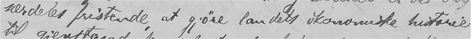særdeles fristende at gjøre landets økonomiske historie
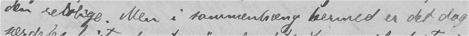den retslige. Men i sammenhæng hermed er det dog
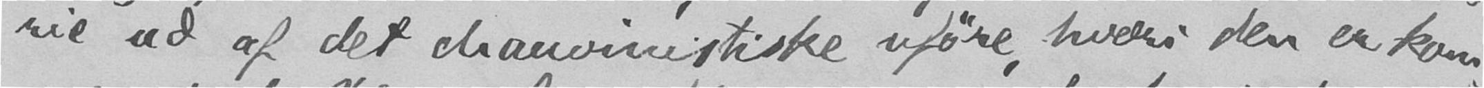rie ud af det chauvinistiske uføre, hvori den er kom-
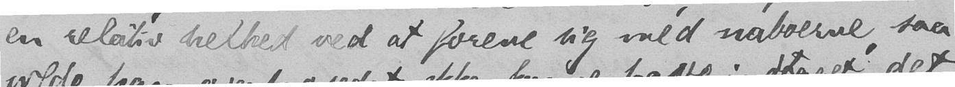en relativ helhed ved at forene sig med naboerne, saa
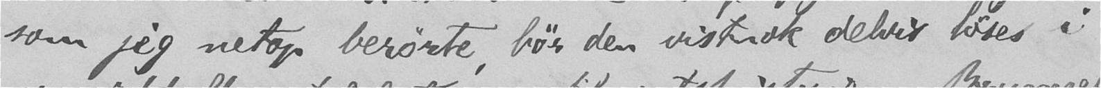som jeg netop berørte, bør den vistnok delvis løses i
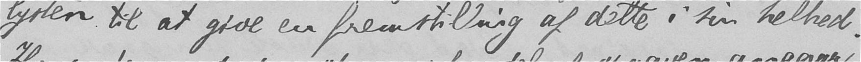lysten til at give en fremstilling af dette i sin helhed.
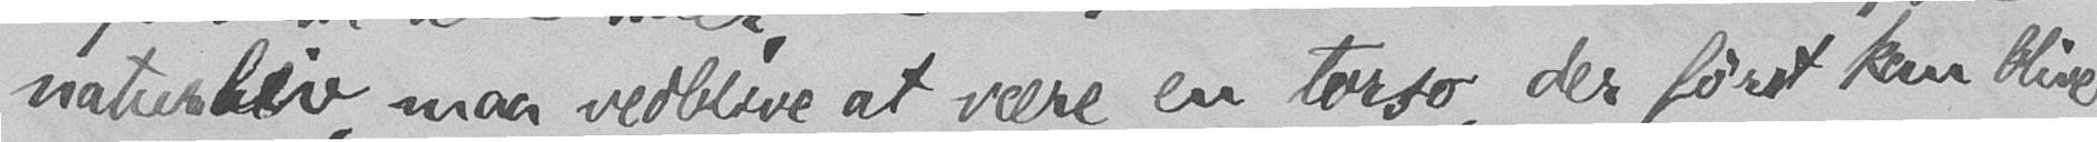naturliv, maa vedblive at være en torso, der først kan blive
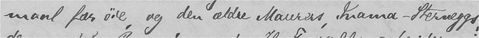maal for øie, og den ældre Maurers, Inama-Sterneggs,
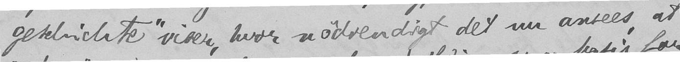geschichte" viser, hvor nødvendigt det nu ansees, at
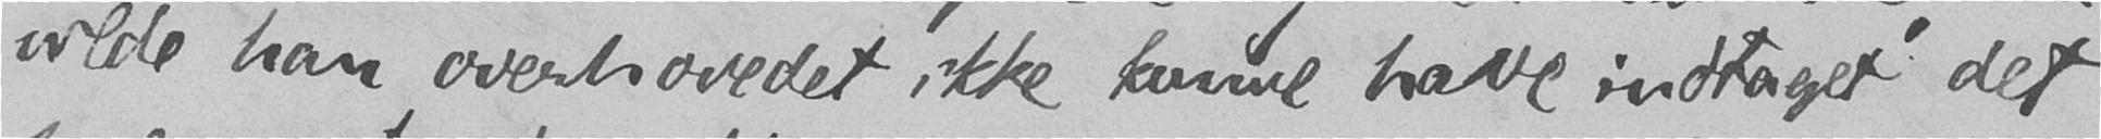vilde han overhovedet ikke kunne have indtaget det
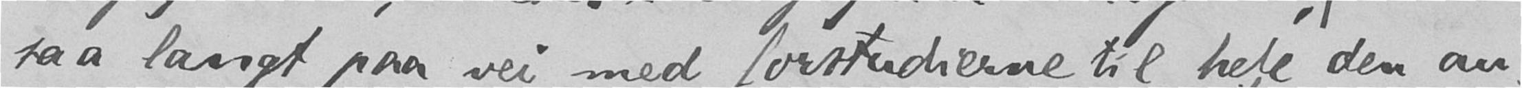saa langt paa vei med forstudierne til hele den an-
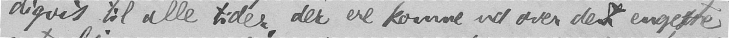digvis til alle tider, der ere komne ud over det engeste
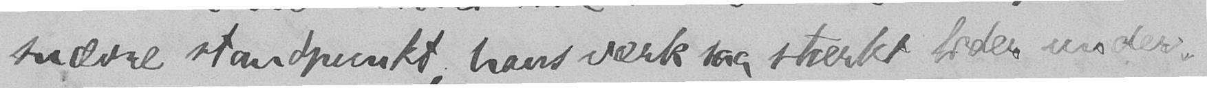snævre standpunkt, hans værk saa stærkt lider under.
